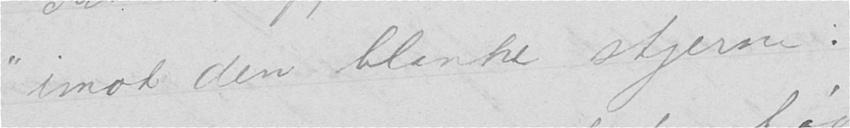"imod den blanke stjerne:
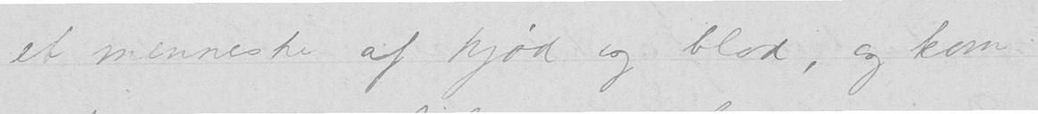et menneske af kjød og blod, og kom
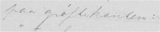paa grøftekanten:
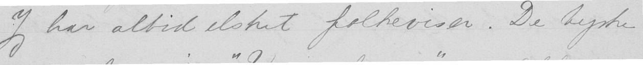Jeg har altid elsket folkeviser. De tyske
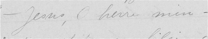"— Jesus, O herre min —
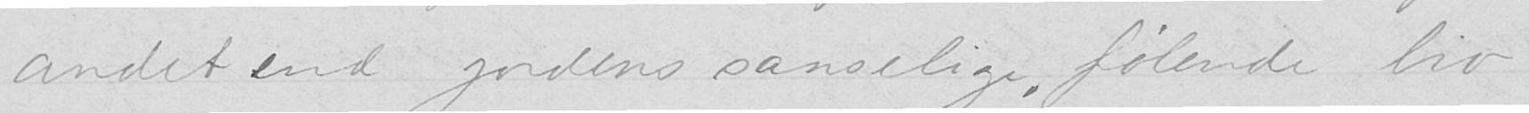andet end jordens sanselige, følende liv
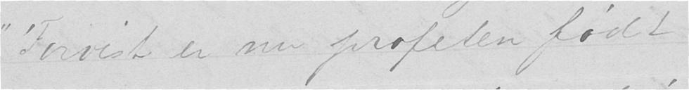"'Forvist er nu profeten født
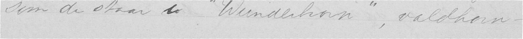som de staar i "Wunderhorn", valdhorn —
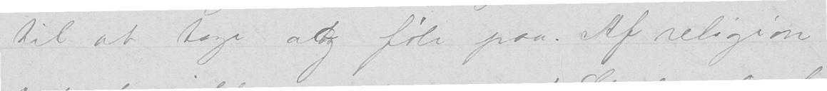til at tage og føle paa. Af religion
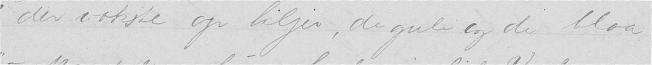"der vokste op liljer, de gule og de blaa
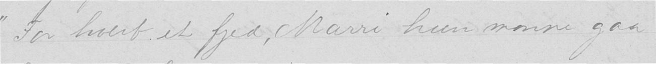"For hvert et fjed, Marri hun monne gaa
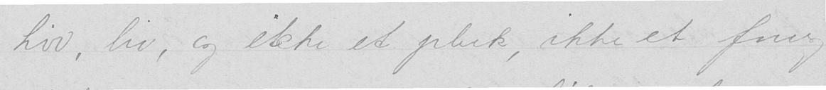Liv, liv, og ikke et pluk, ikke et fnug
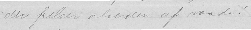"der frelser alverden af vaade!"
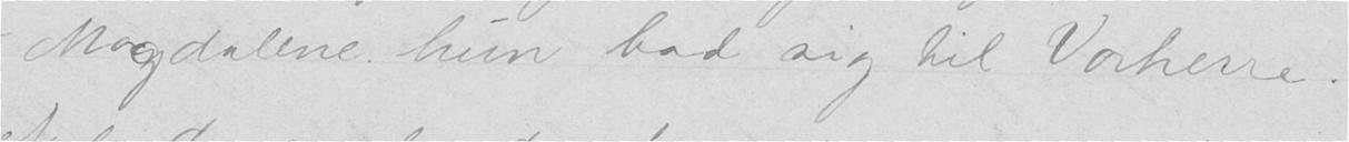"— Magdalene hun bad sig til Vorherre."
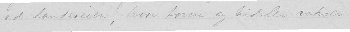ad landeveien, hvor torne og tidsler vokser
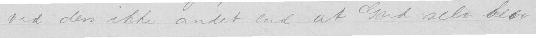ved den ikke andet end at Gud selv blev
